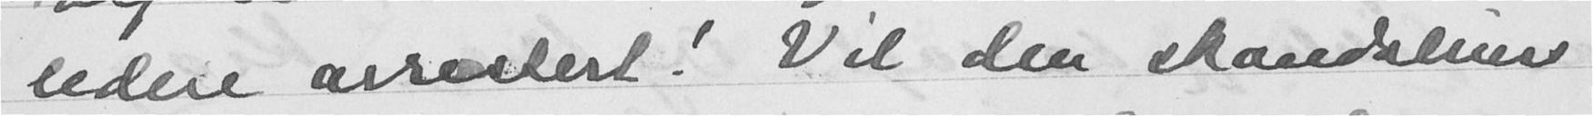ledere arrestert. Vil den skandalens
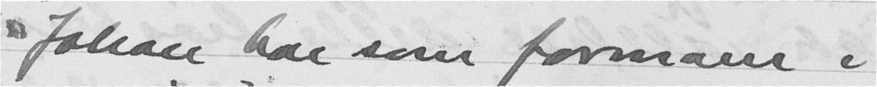Johan har som formann i
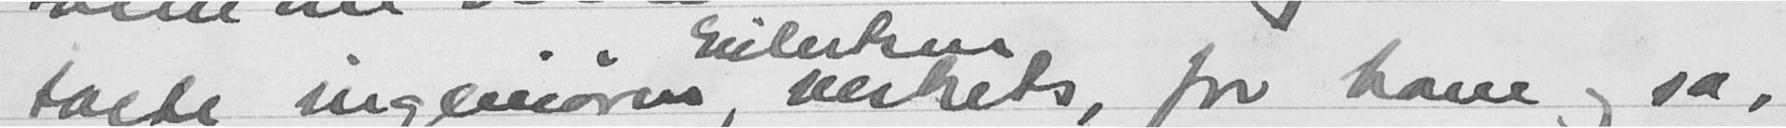talte ingeniøren Eilertsen, verkets, for ham og sa,
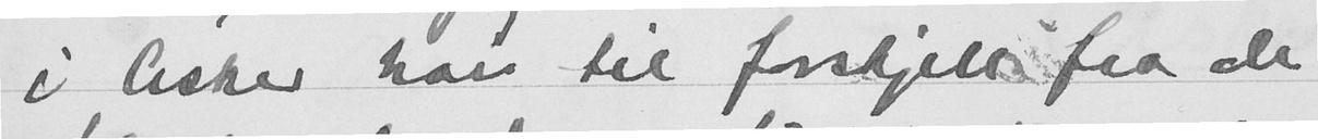i Asker har til forskjell fra de
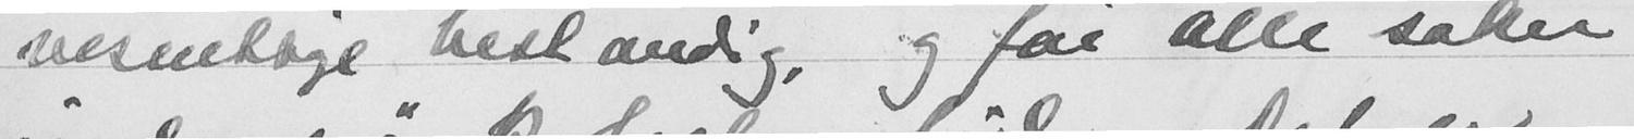vesentlige helt andig, og får alle saker
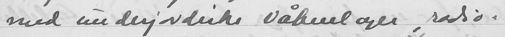med underjordiske Våbenlager, radio-
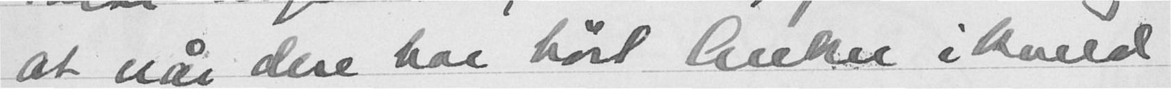at når dere har hørt Anker i kveld
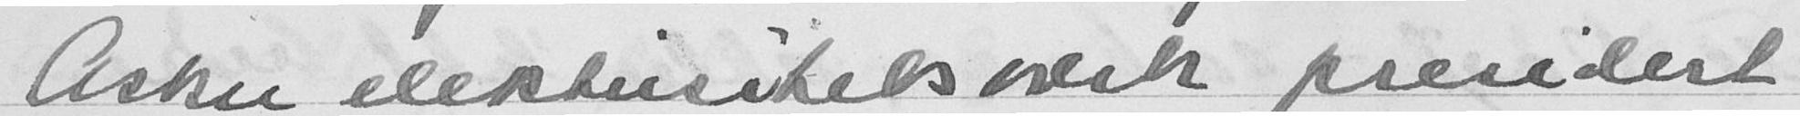Asker elektrisitetsværk presidert
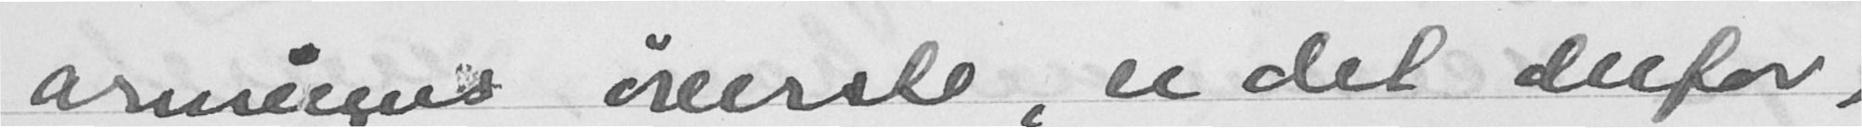armenns øverste, er det derfor,
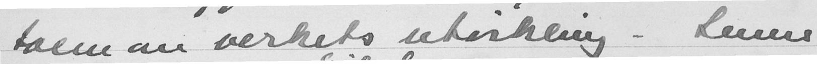talen om verkets utvikling. Senere
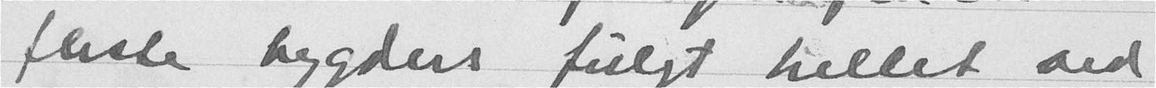fleste bygders fulgt hellet ved
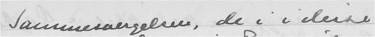Sammesvergelsen, de i i disse
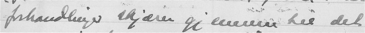forhandlinger skjærer gjennom til det
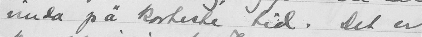unda på korteste tid. Det er
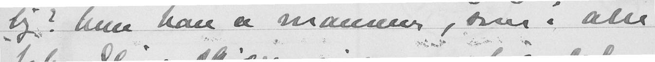lig? Men han er mannen, som i alle
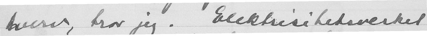verv, tror jeg. Elektrisitetsverket
